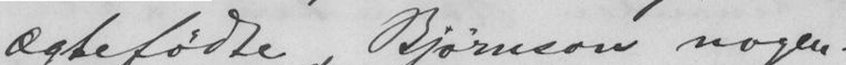ægtefødte, Bjørnson nogen-
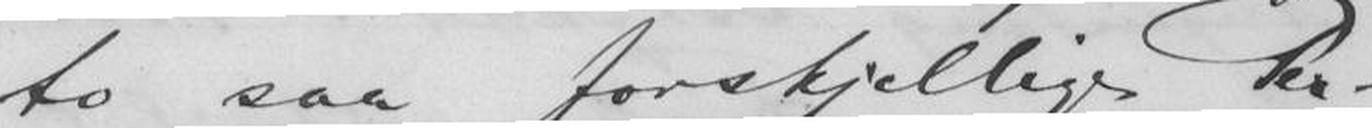to saa forskjellige Per-
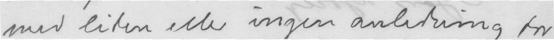med liten eller ingen anledning for
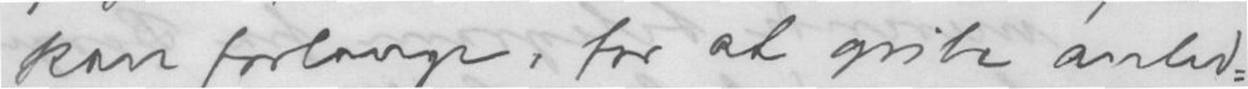kan forlange, for at gribe anled-
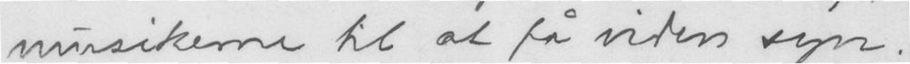musikerne til at få viden syn.
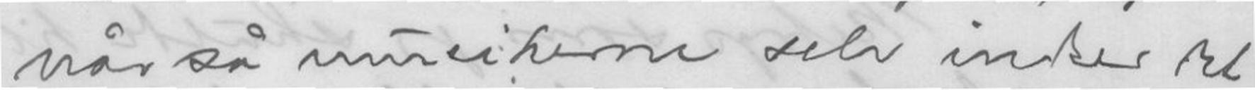når så musikerne selv indser det
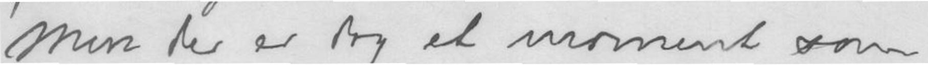Men der er dog et moment som
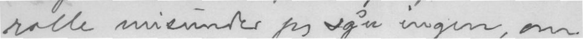rolle misunder jeg sgu ingen, om
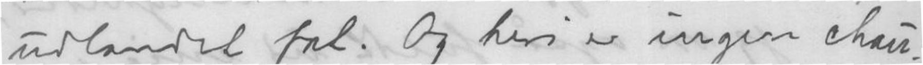udlandet fat. Og heri er ingen chau-
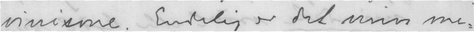vinisme. Endelig er det min me-
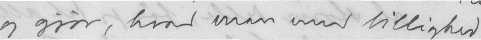og gjør, hvad man med billighed
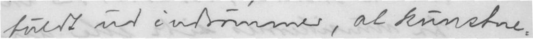fuldt ud indrømmer, at kunstne-
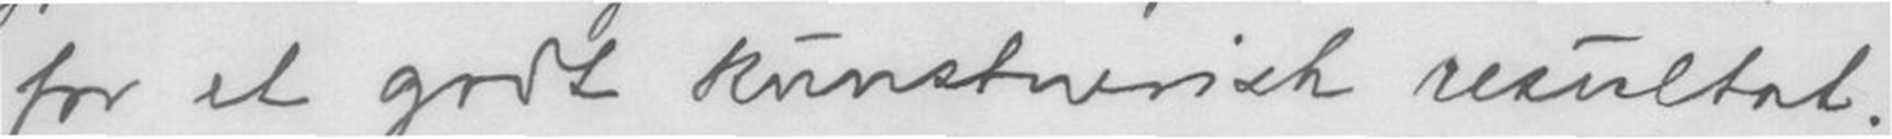for et godt kunstnerisk resultat.
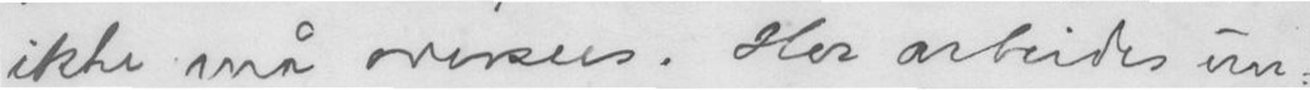ikke må oversees. Her arbeides un-
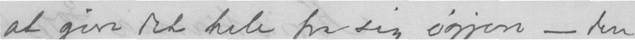at give det hele fra sig igjen - den
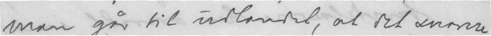man går til udlandet, at det snarere
